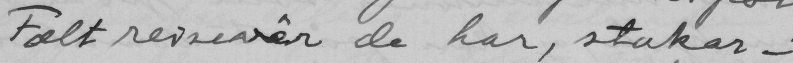Fælt reisevêr de har, stakar -
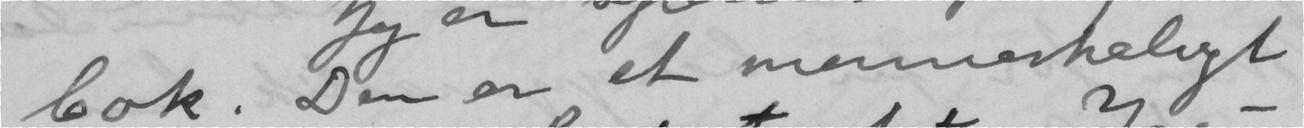bok. Den er et mennerkeligt
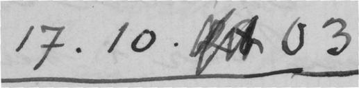17.10. 03
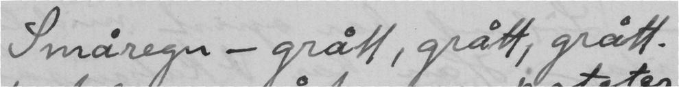Småregn - grått, grått, grått.
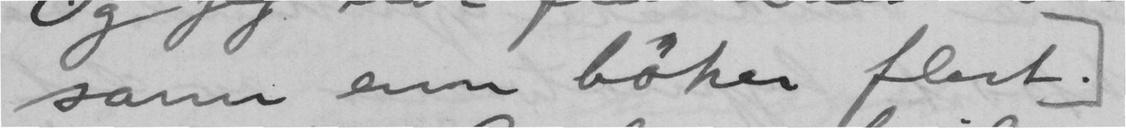sann enn bøker flest.
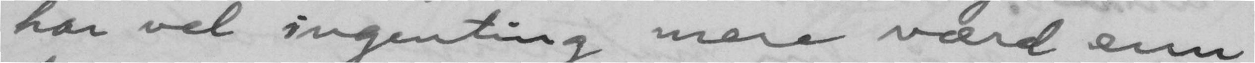har vel ingenling mere værd enn
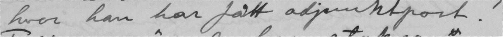hvor han har fått adjunktpost!
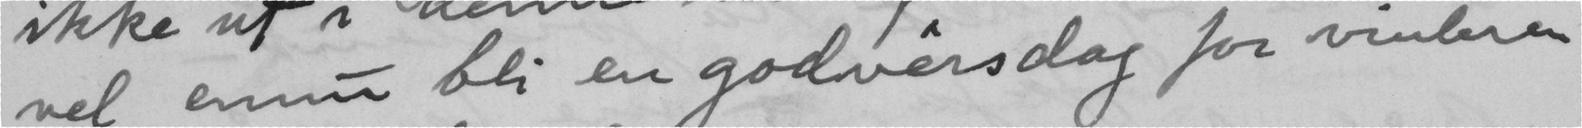vel ennu bli en godvêrsdag for vinteren
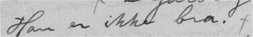Han er ikke bra. x
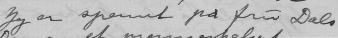Jeg er spennt på fru Dals
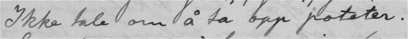Ikke tale om å ta opp poteter.
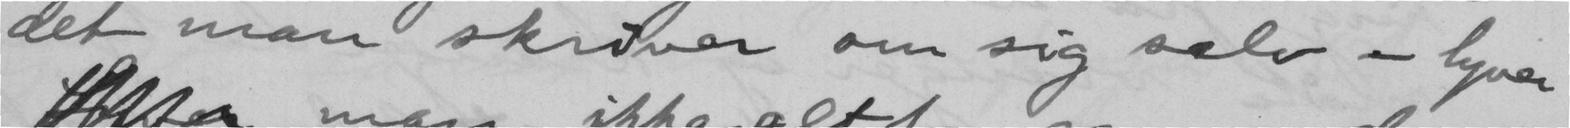det man skriver om sig selv - Lyver
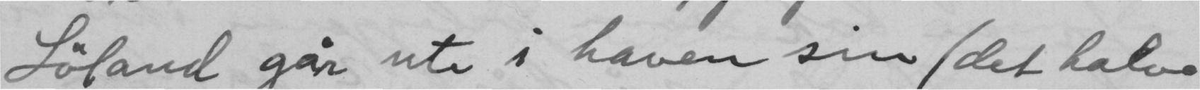Løland går ute i haven sin (det halve
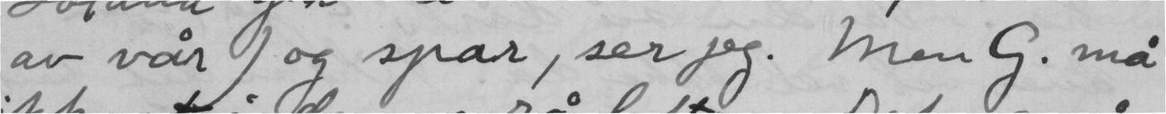av vår) og spar, ser jeg. Men G. må
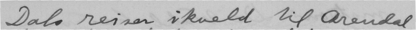Dals reiser ikveld til Arendal
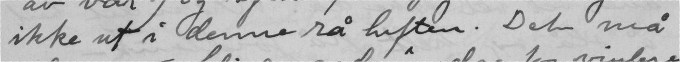ikke ut i denne rå luften. Det må
